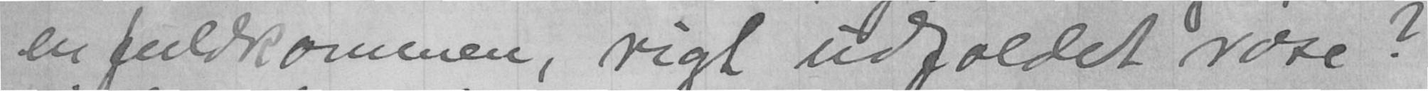en fuldkommen, rigt udfoldet rose?
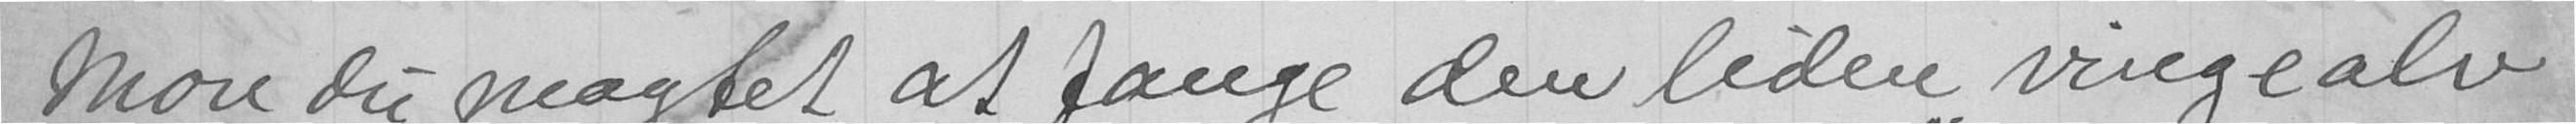Mon du magtet at fange den liden vingealv
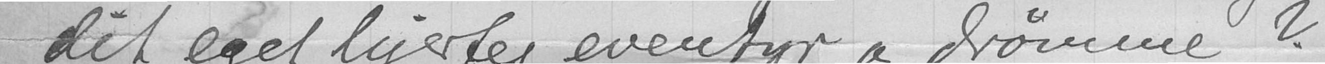- dit eget hjertes eventyr og drømme?
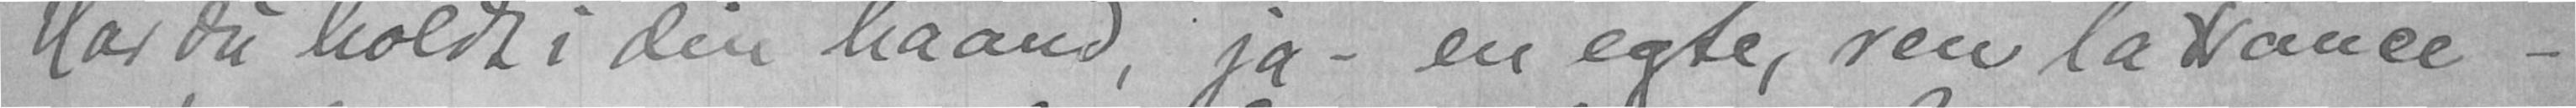Har du holdt i den haand, ja - en egte, ren la France -
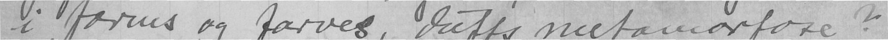i forms og farves, dufts metamorfose?
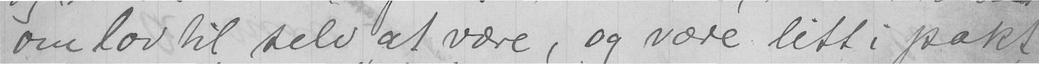om lov til selv at være, og være litt i pakt
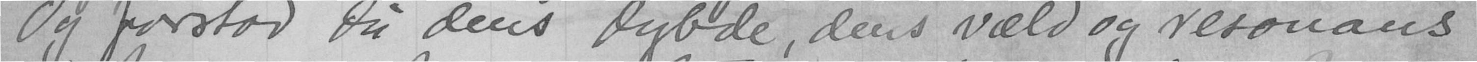Og forstod du dens dybde, dens væld og resonans
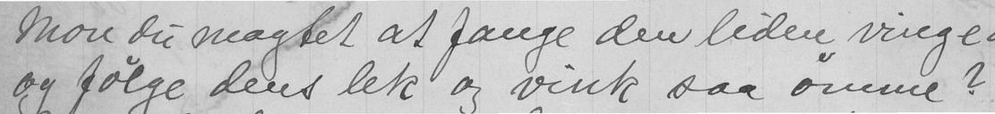og følge dens lek og vink saa ømme?
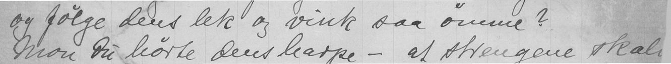Mon du hørte dens harpe - at strengene skal
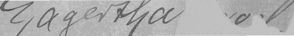Lagertha Broch
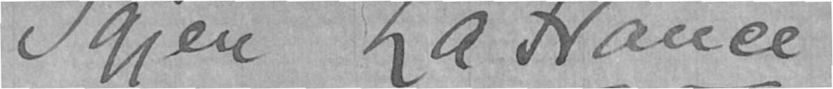Igjen La France!
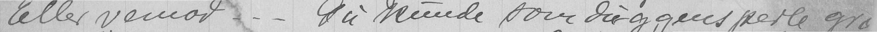Eller vemod - - - Du kunde som du og gens perle græ
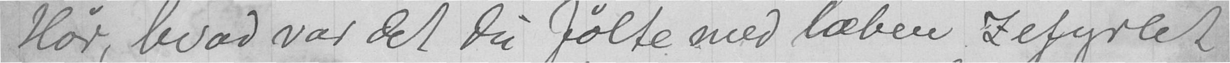Hør, hvad var det du følte med læben, Zefyrlet
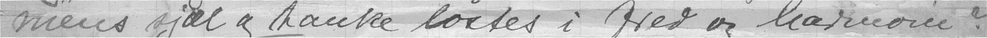mens sjæl og tanke løstes i fred og harmonie?
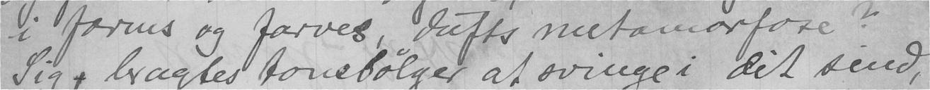Sig + bragtes tonebølger at svinge i dit sind,
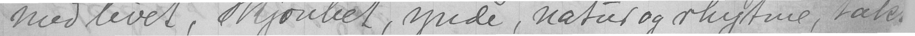med livet, skjønhet, ynde, natur og rhytme, tak.
